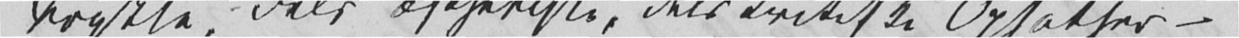trykte, dels æsthetiske, dels kritiske Opsatser –
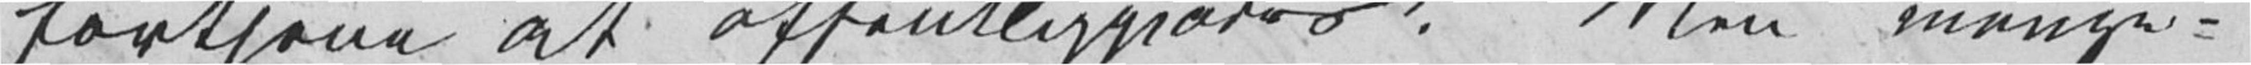fortjene at offentliggjøres. Men mange¬
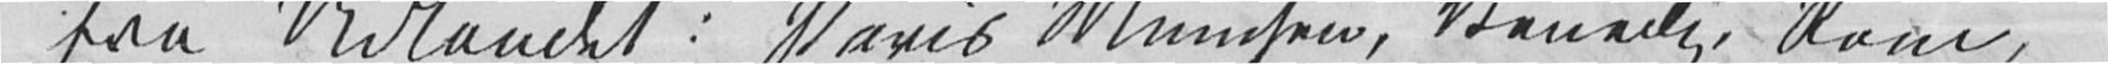fra Udlandet: Paris München, Venedig, Rom,
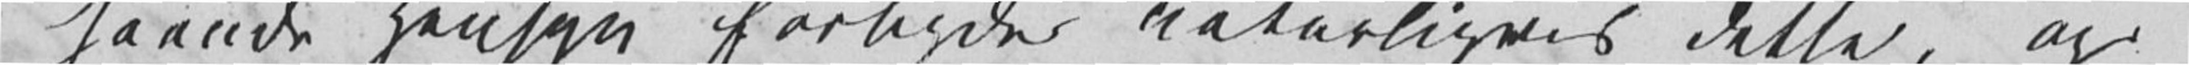haande Hensyn forbyder naturligvis dette, og
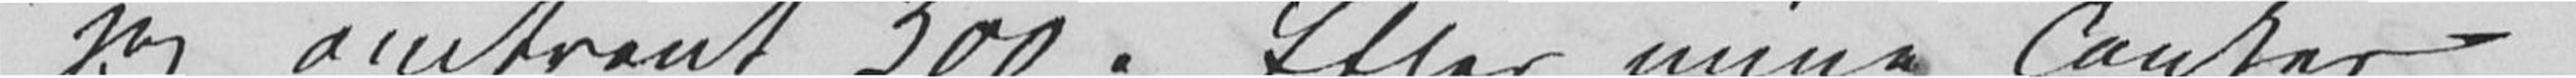jeg omtrent 300. Efter mine Tanker
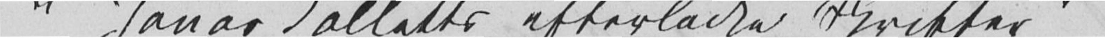«Jonas Colletts efterladte Skrifter»
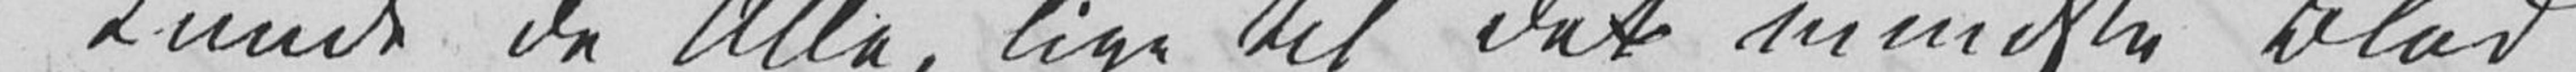kunde de Alle, lige til det mindste Blad
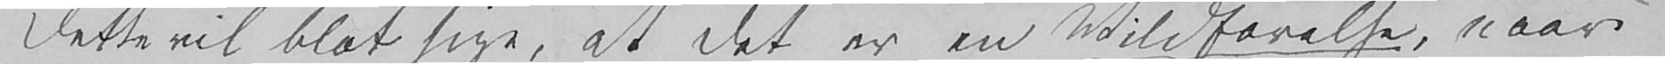Dette vil blot sige, at det er en Vildfarelse, naar
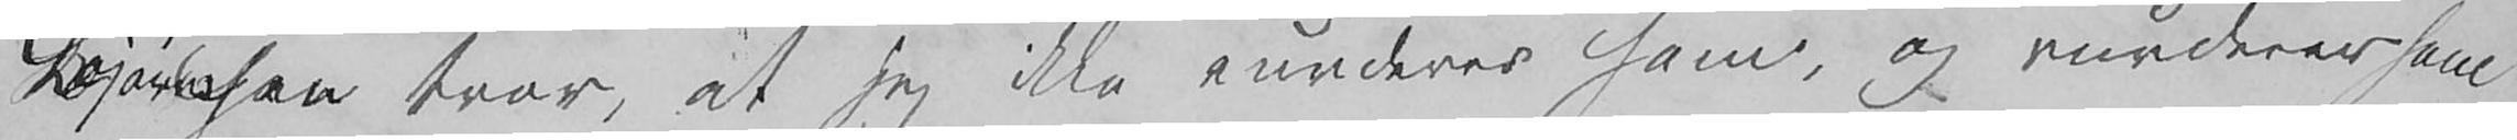Bjørnson tror, at jeg ikke vurderer ham, og vurderer ham
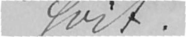høit.
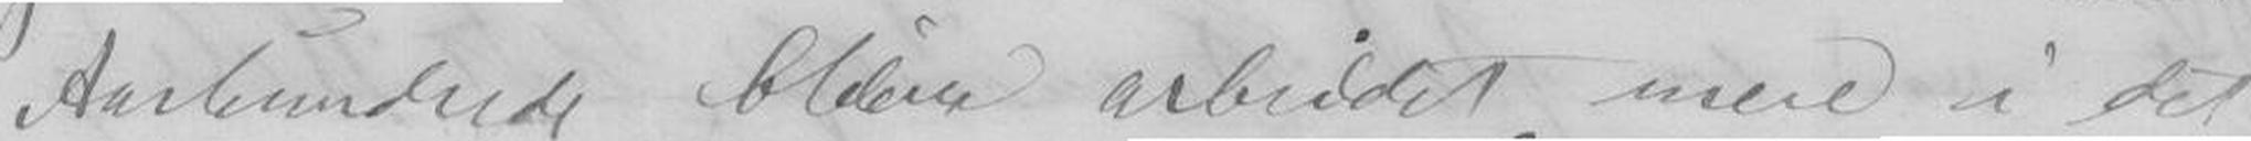Aarhundrede bliver arbeidet mere i det
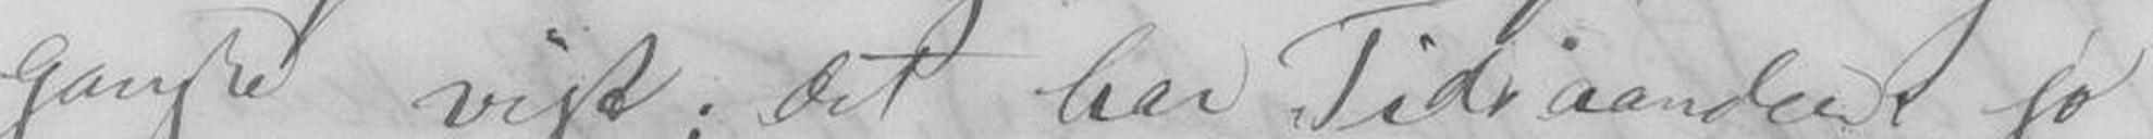ganske vist; det har Tidsaanden jo
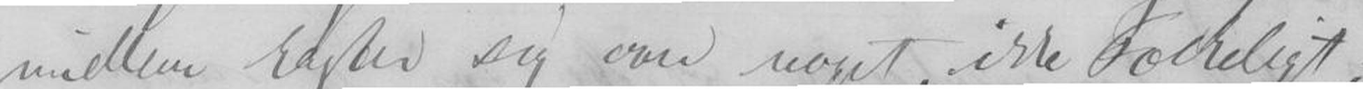imellem kaster sig over noget ikke Folkeligt;
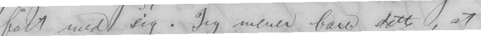ført med sig. Jeg mener bare dette, at
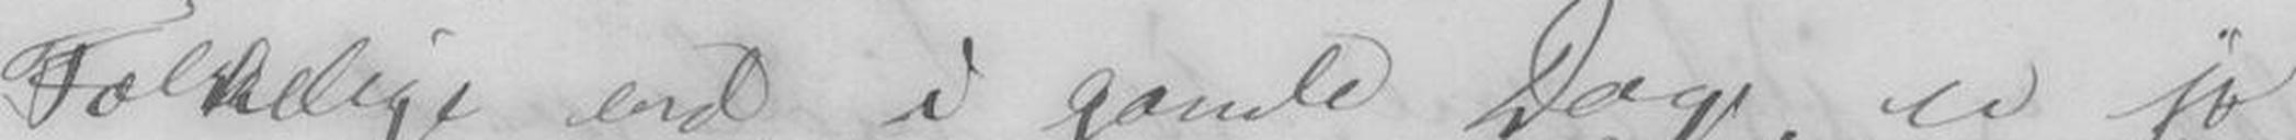Folkelige end i gamle Dage, er jo
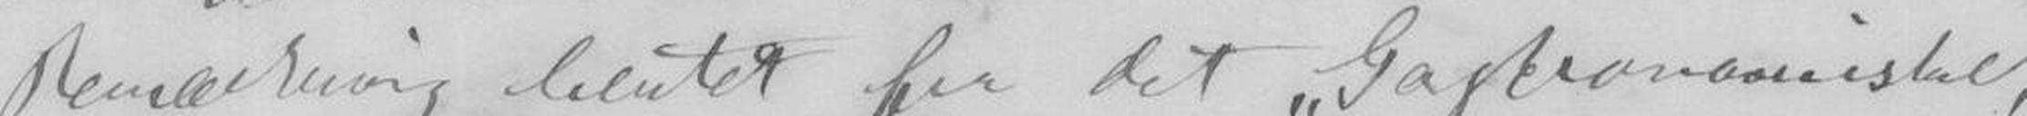Bemærkning hentet for det Gastronomiske,
